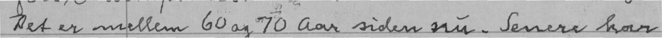Det er mellem 60 og 70 Aar siden nu. Senere har
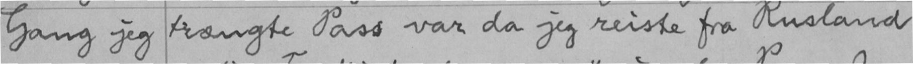Gang jeg trængte Pass var da jeg reiste fra Rusland
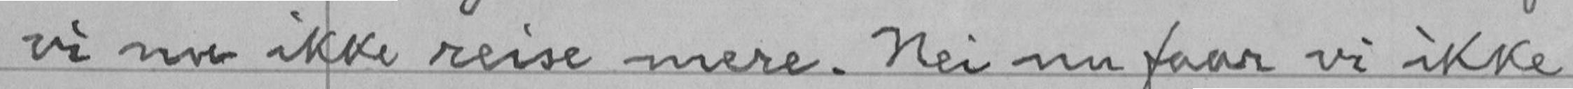vi nu ikke reise mere. Nei nu faar vi ikke
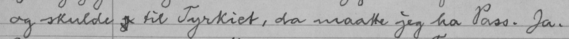og skulde j til Tyrkiet, da maatte jeg ha Pass. Ja.
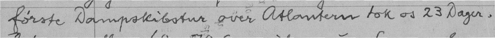første Dampskibstur over Atlantern tok os 23 Dager.
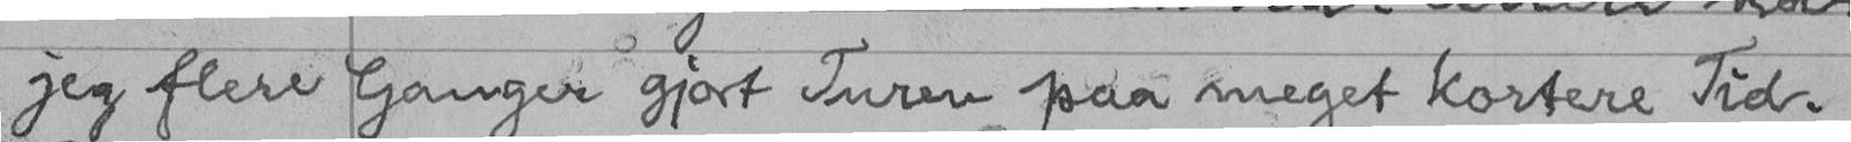jeg flere Ganger gjort Turen paa meget kortere Tid.
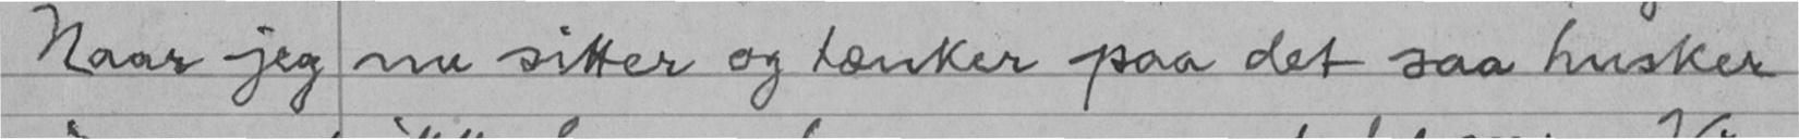Naar jeg nu sitter og tænker paa det saa husker
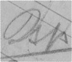Dsp
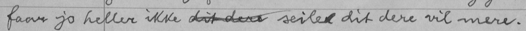faar jo heller ikke dit dere seiler dit dere vil mere.
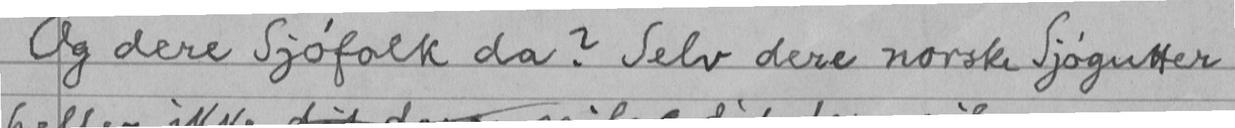Og dere Sjøfolk da? Selv dere norske Sjøgutter
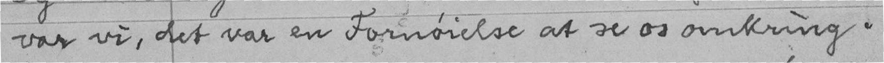var vi, det var en Fornøielse at se os omkring.
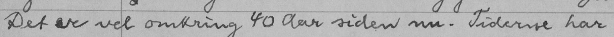Det er vel omkring 40 Aar siden nu. Tiderne har
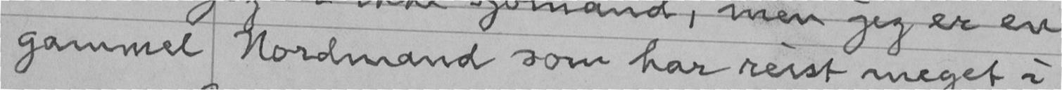gammel Nordmand som har reist meget i
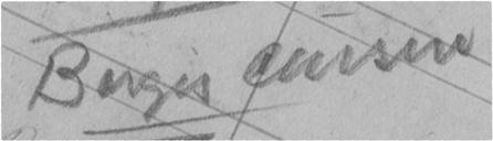Bugis cuisin
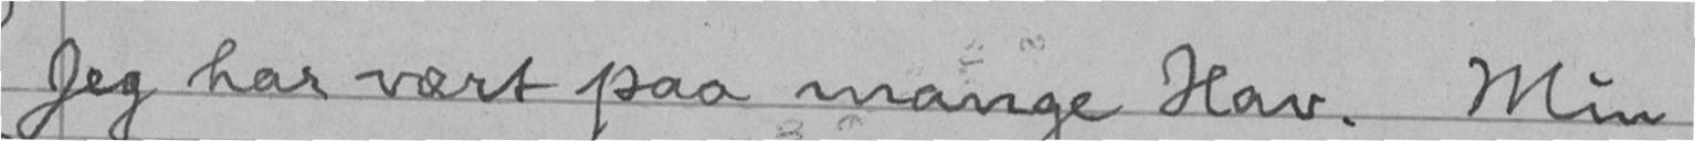Jeg har vært paa mange Hav. Min
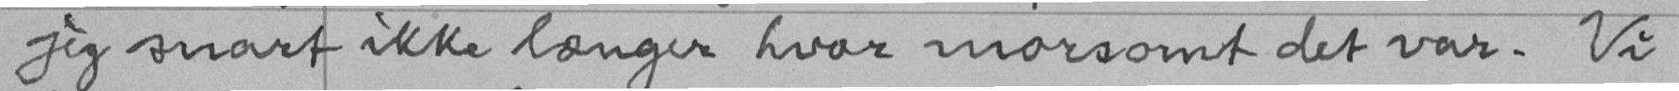jeg snart ikke længer hvor morsomt det var. Vi
